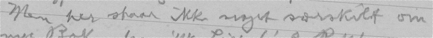Men her staar ikke noget særskilt om
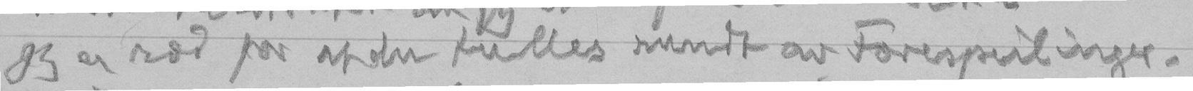jeg er ræd for at du tulles rundt av Forespeilinger.
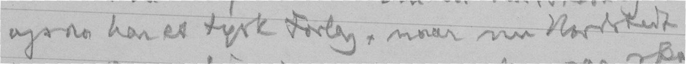ogsaa har et tysk Forlag, naar nu Nordstedt
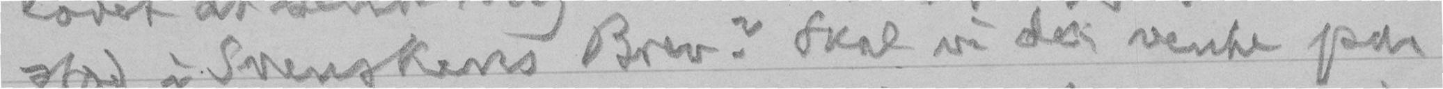stod i Svenskens Brev? Skal vi da vente paa
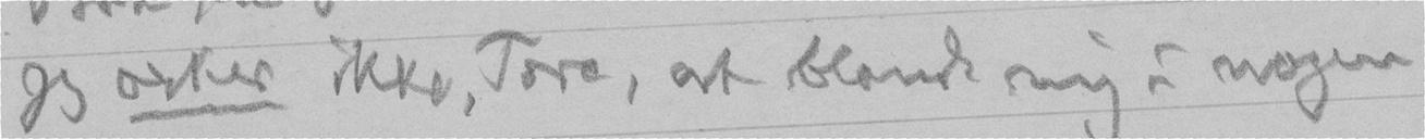Jeg orker ikke, Tore, at blande mig i nogen
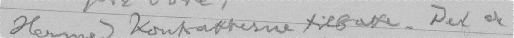Hermed Kontrakterne tilbake. Det er
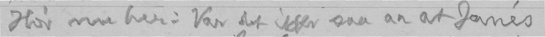Hør nu her: Var det ikke saa an at Janés
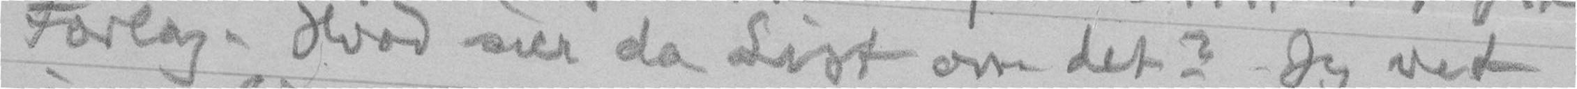Forlag. Hvad sier da List om det? Jeg vet
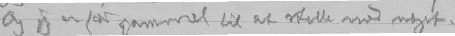Og jeg er for gammel til at stelle med noget.
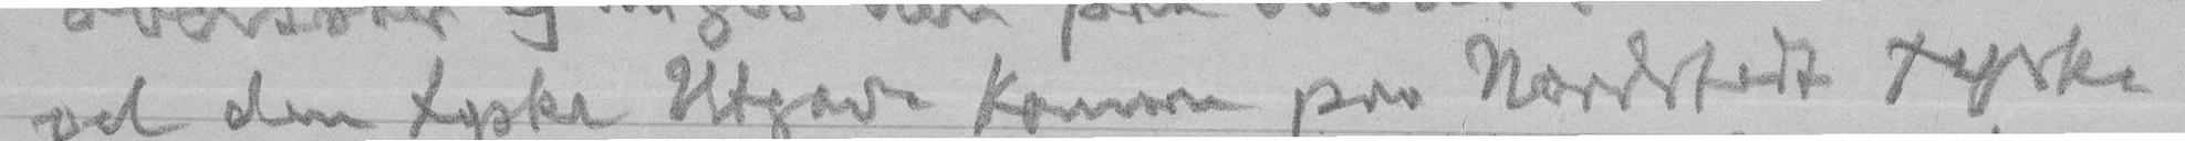vel den tyske Utgave komme paa Nordstedt tyske
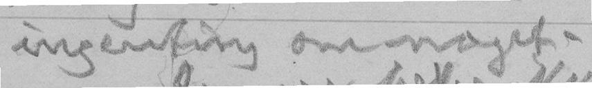ingenting om noget.
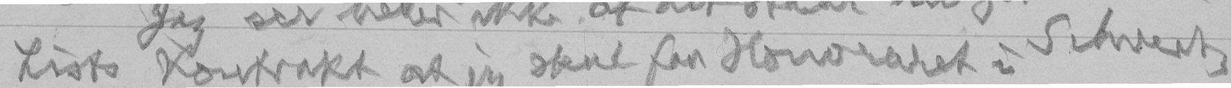Lists Kontrakt at jeg skal faa Honoraret i Schveitz
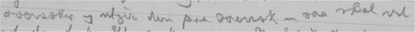oversætter og utgir den paa svensk - saa skal vel
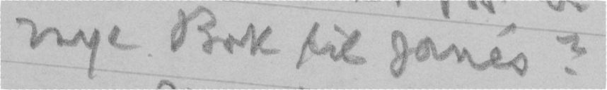nye Bok til Janés?
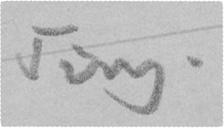Ting.
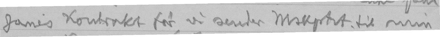Janés Kontrakt før vi sender Mskptet. til min
